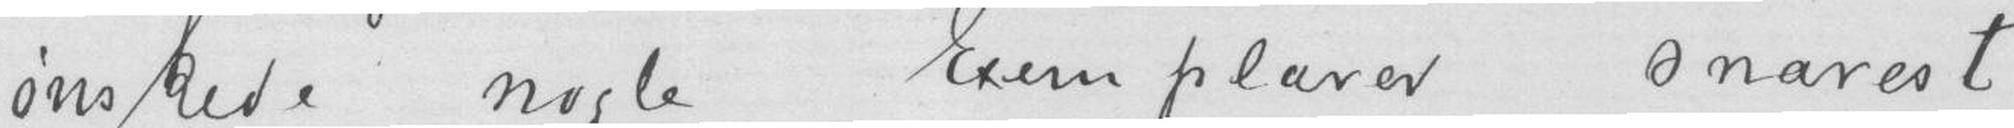ønskede nogle Exemplarer snarest
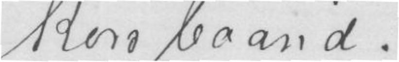korsbaand.
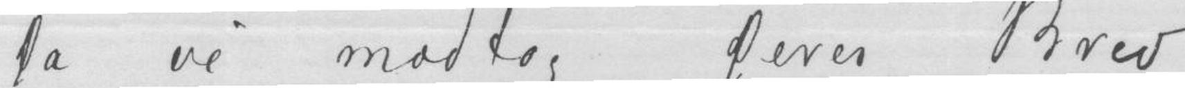Da vi modtog Deres Brev
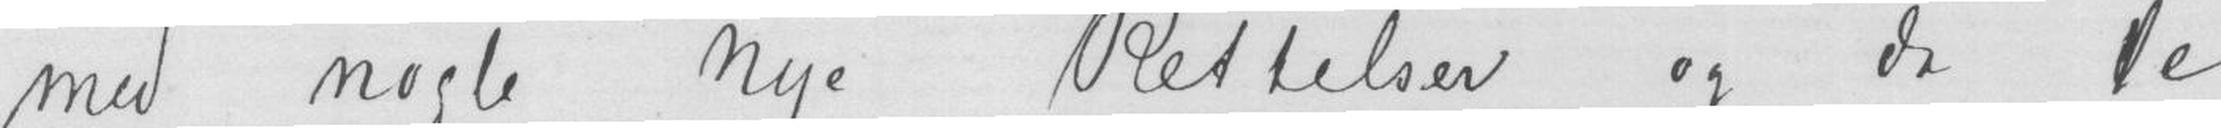med nogle Nye Rettelser og da De
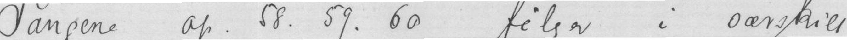Sangene op. 58.59. 60 følger i Særskill
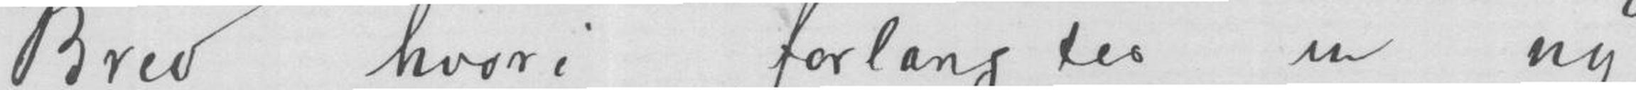Brev hvori forlangtes en ny
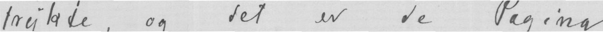trykte, og det er de Pagina
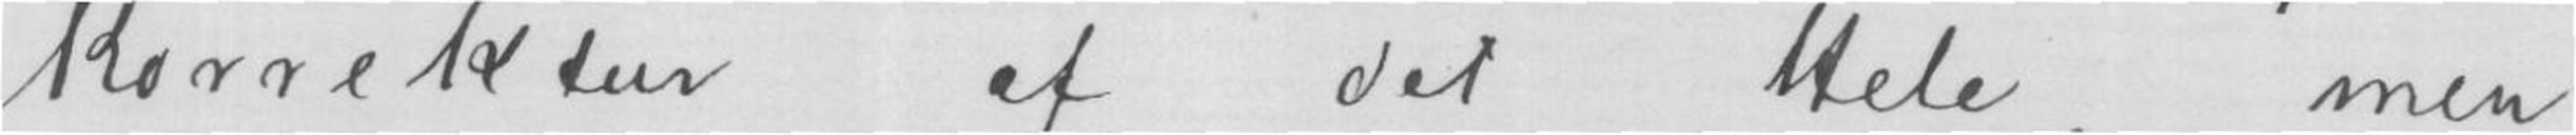Korrektur af det Hele, men
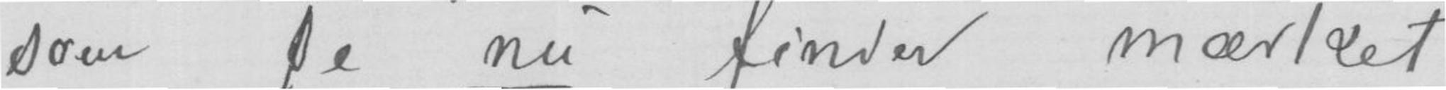som De nu finder mærket
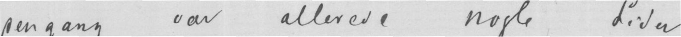dengang var allerede nogle Lider
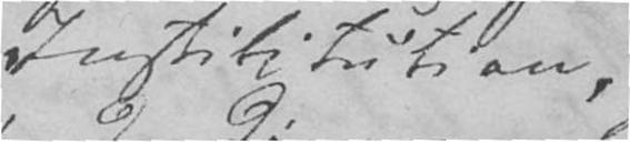Institution.
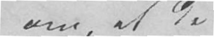om at de
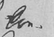Cra-
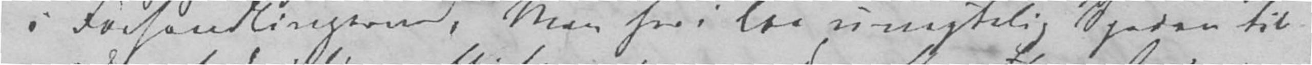i Forhandlingerne. Men heri laa unegtelig Sporen til
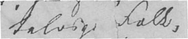talrige Folk,
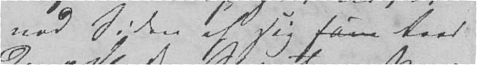ved siden af sig Raad-
